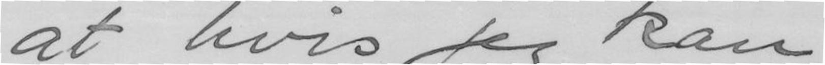at hvis jeg kan
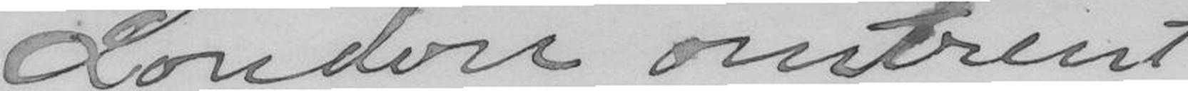London omtrent
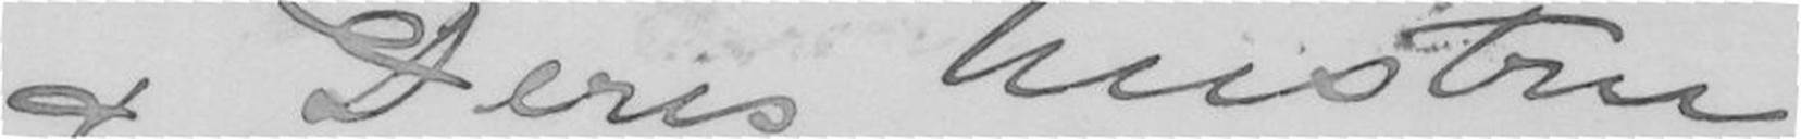& Deres hustru
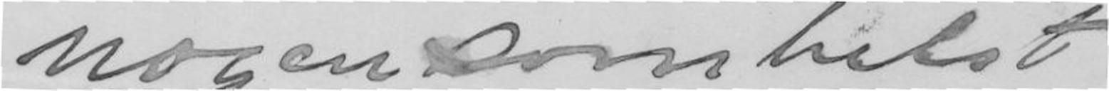nogensomhelst
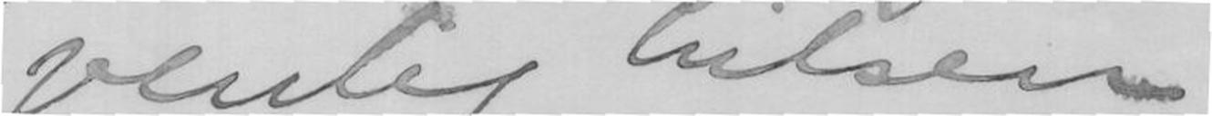venlig hilsen
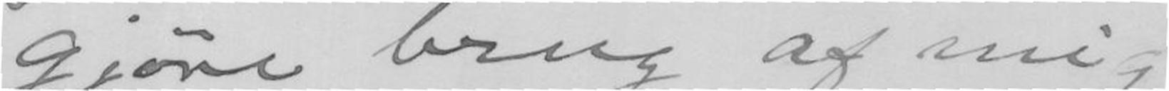gjøre brug af mig
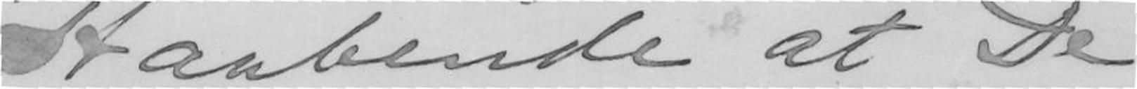Haabende at De
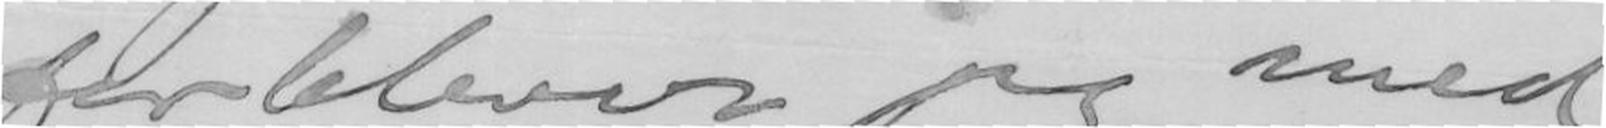forbliver jeg med
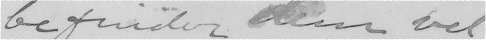befinder dem vel
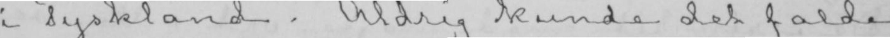i Tyskland. Aldrig kunde det falde
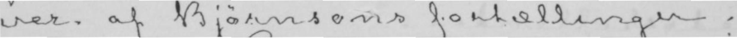ver af Bjørnsons fortællinger.
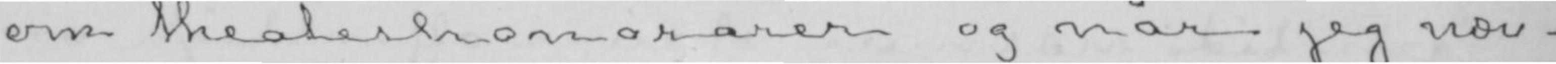om theaterhonorarer og når jeg næv-
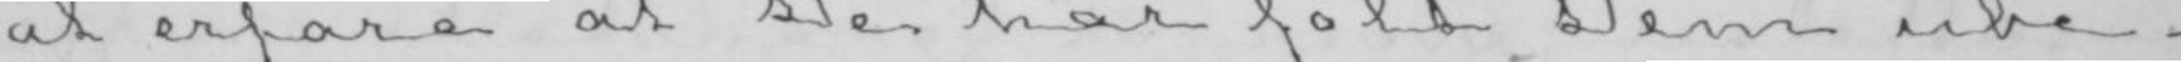at erfare at De har følt Dem ube-
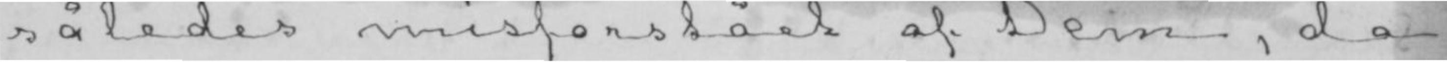således misforstået af Dem, da
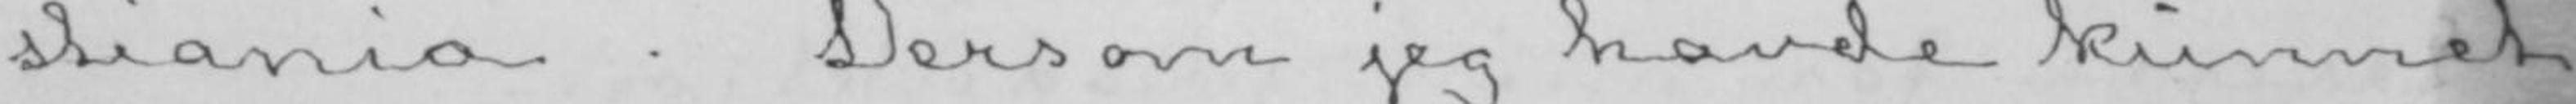stiania. Dersom jeg havde kunnet
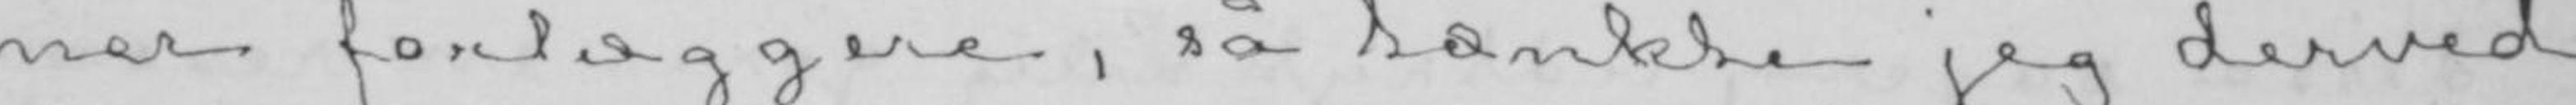ner forlæggere, så tænkte jeg derved
